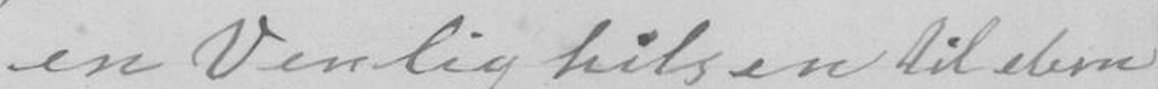en Venlig hilsen til dere
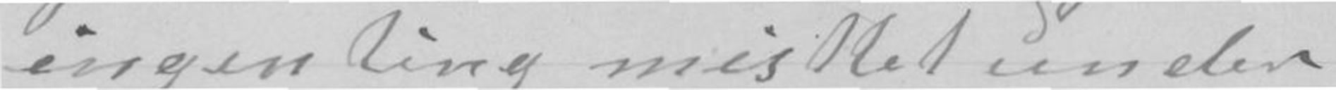ingenting misttet under
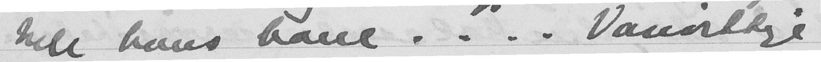hele hans bane.... Vanvittige
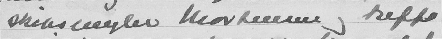skibsmegler Mortensen og treffe
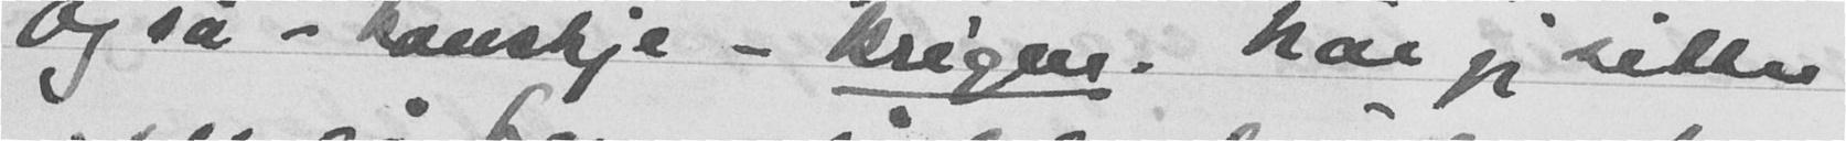Og så - kanskje - krigen. Når jeg sitter
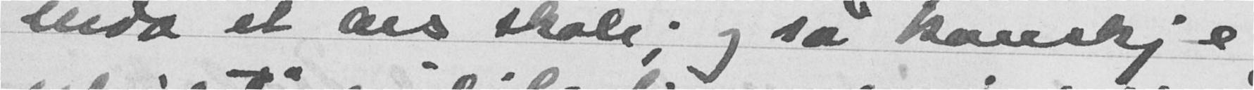enda et års skole; og så kanskje
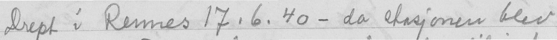Drept i Rermes 17. 6. 40 - da stasjonen blev
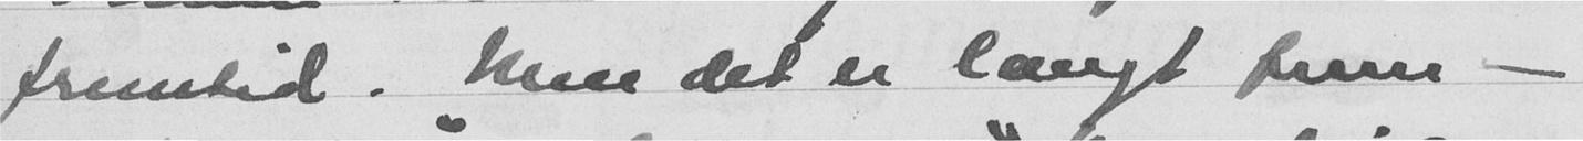fremtid. Men det er langt frem -
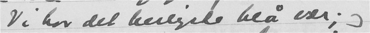Vi har det herligste blå vær; og
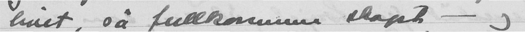livet, så fullkommen skapt - og
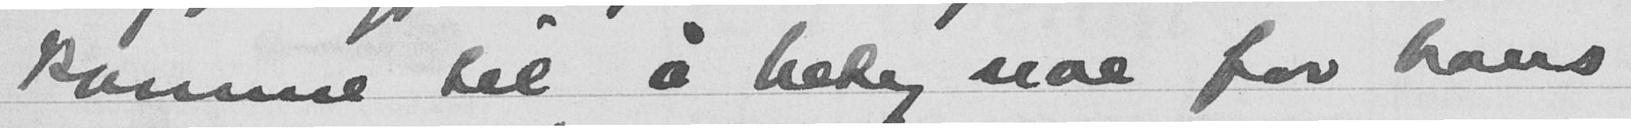komme til å bety noe for hans
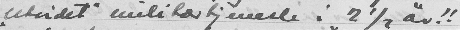utvidet militærtjeneste i 2 1/2 år!!
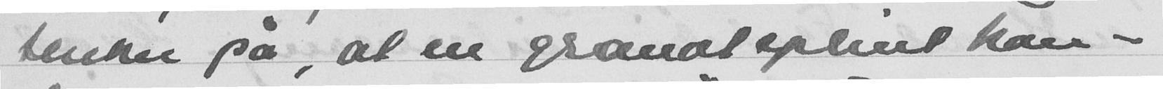tenker på, at en granatspling kan-
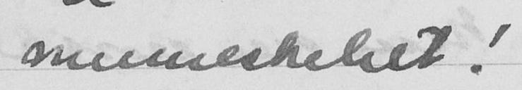menneskehet!
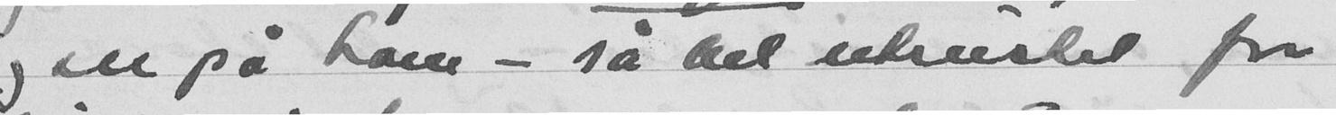og ser på ham - så vel utrustet for
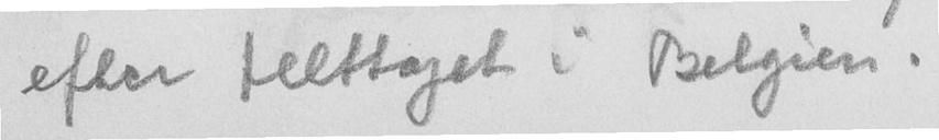efter felttoget i Belgien.
